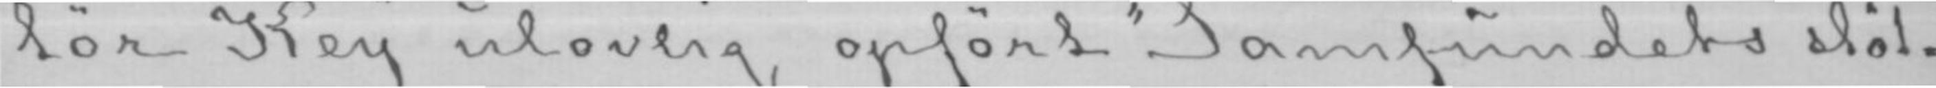tør Key ulovlig opført «Samfundets støt-
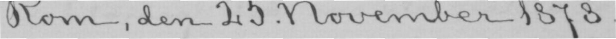Rom, den 25. November 1878.
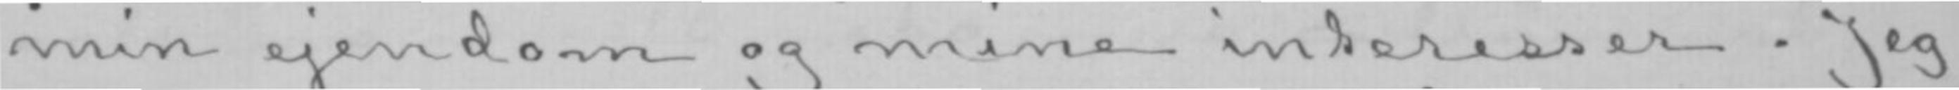min ejendom og mine interesser. Jeg
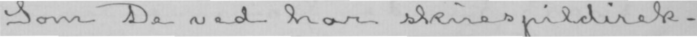Som De ved har skuespildirek-
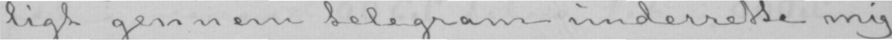ligt gennem telegram underrette mig
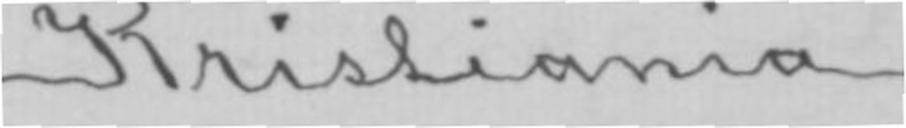Kristiania.
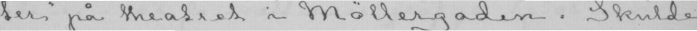ter» på theatret i Møllergaden. Skulde
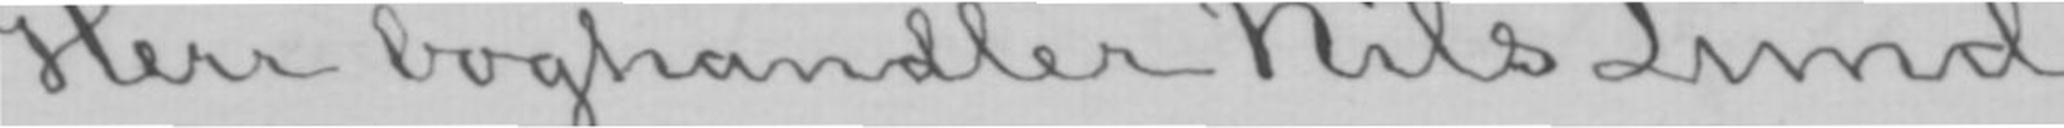Herr boghandler Nils Lund.
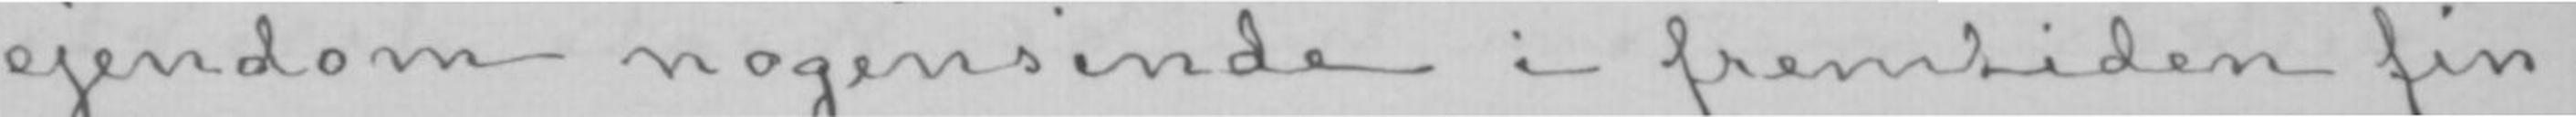ejendom nogensinde i fremtiden fin-
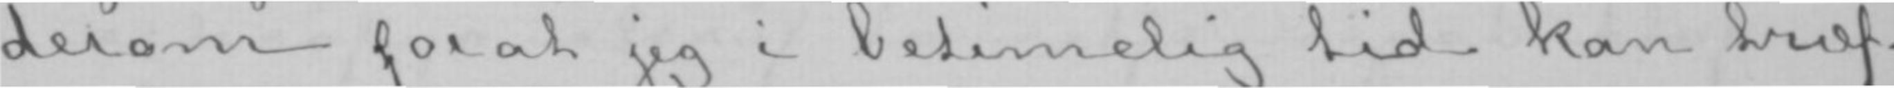derom for at jeg i betimelig tid kan træf-
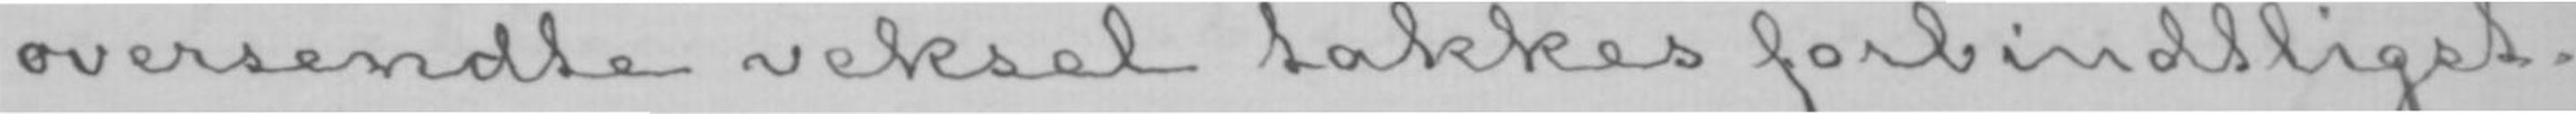oversendte veksel takkes forbindtligst.
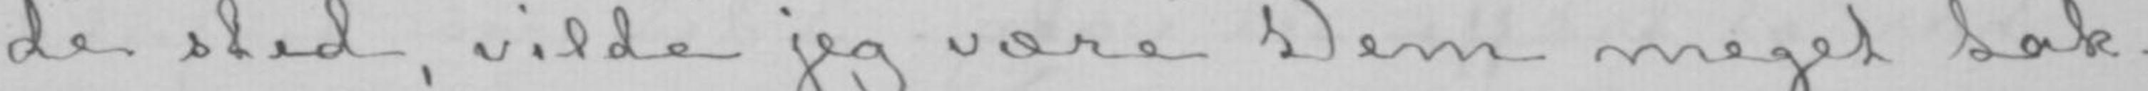de sted, vilde jeg være Dem meget tak-
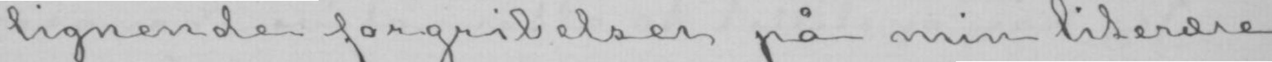lignende forgribelser på min literære
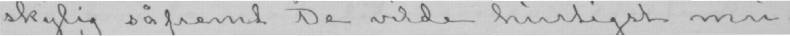skylig såfremt De vilde hurtigst mu-
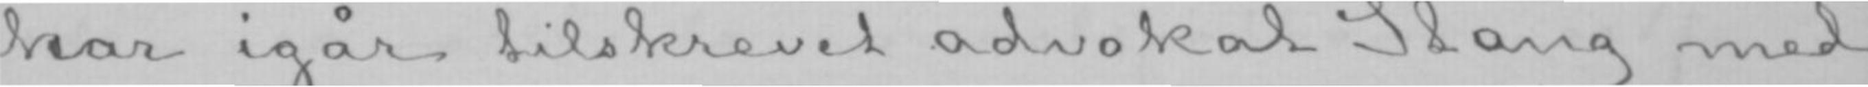har igår tilskrevet advokat Stang med
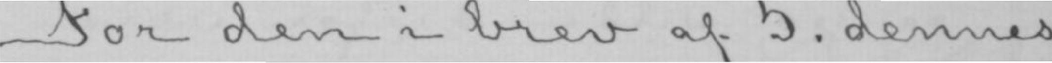For den i brev af 5. dennes
